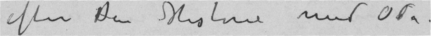efter Din Historie med Oda
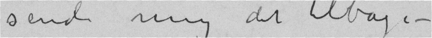sende mig det tilbage –
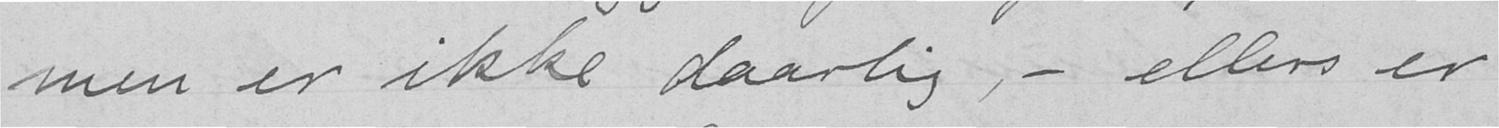men er ikke daarlig, - ellers er
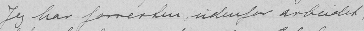Jeg har forresten, udenfor arbeidet,
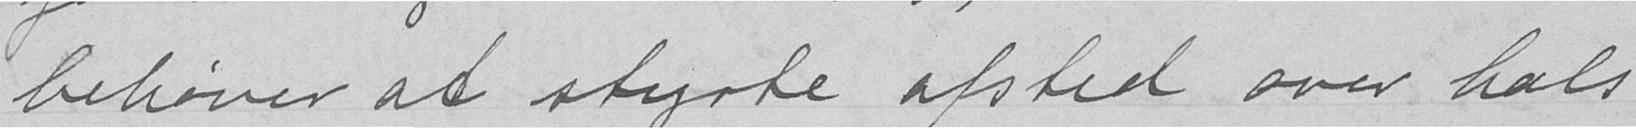behøver at styrte afsted over hals
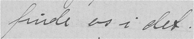finde os i det.
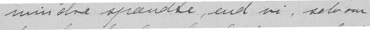mindre spændte, end vi, selv om
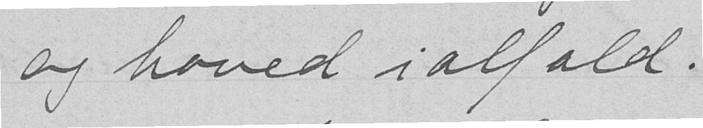og hoved ialfald.
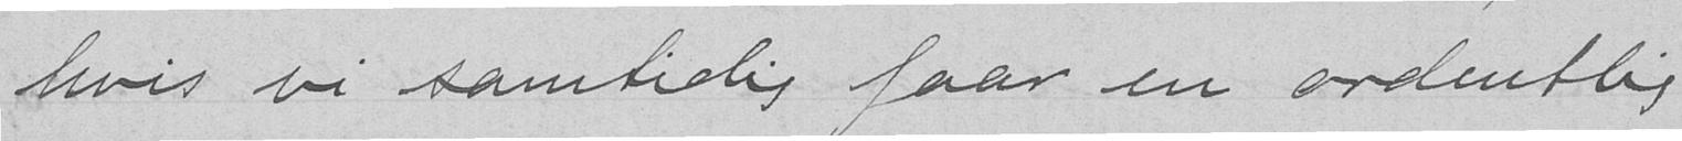hvis vi samtidig faar en ordentlig
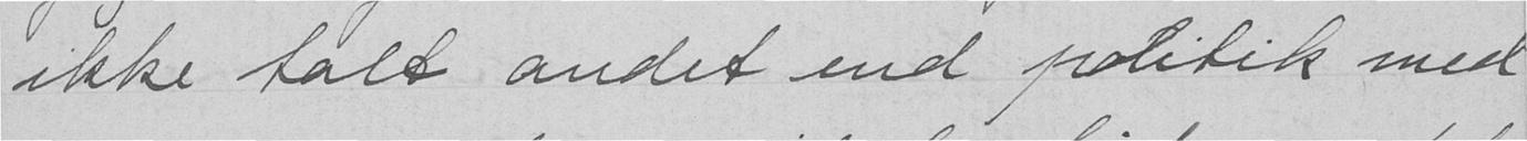ikke talt andet end politik med
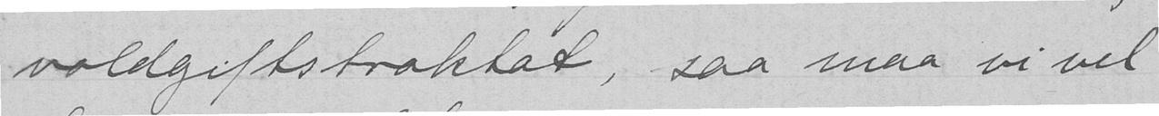voldgiftstraktat, saa maa vi vel
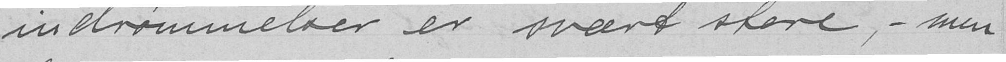indrømmelser er svært store, - men
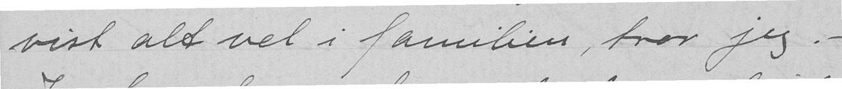vist alt vel i familien, tror jeg. -
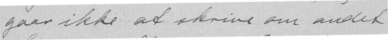gaar ikke at skrive om andet
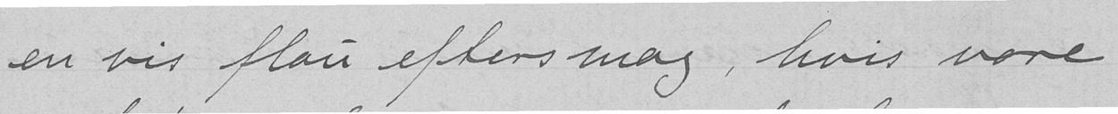en vis flau eftersmag, hvis vore
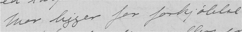Mor ligger for forkjølelse,
